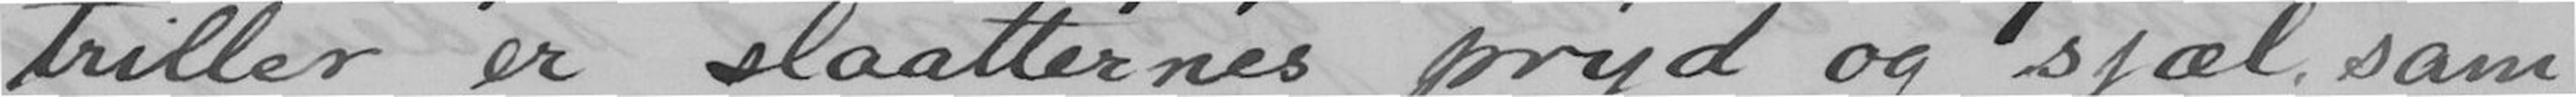triller er slaatternes pryd og sjæl, sam-
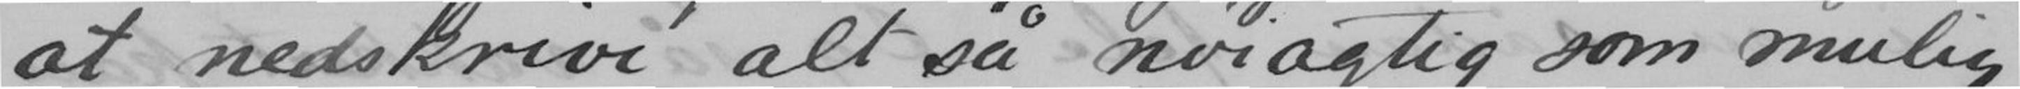at nedskrive alt så nøiagtig som mulig.
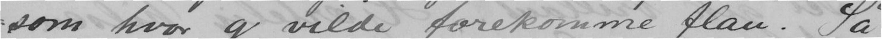som hvor g vilde forekomme flau. Så
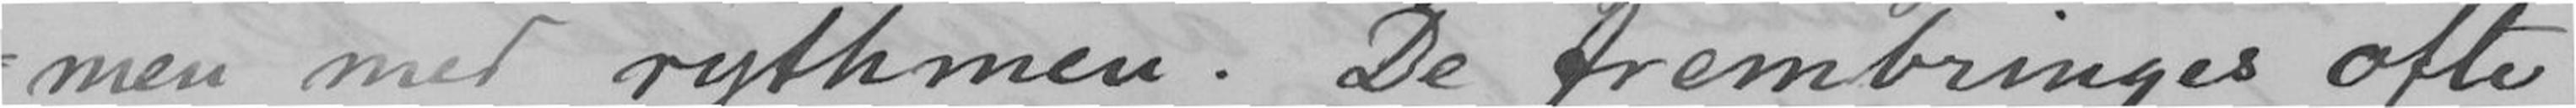men med rythmen. De frembringes ofte
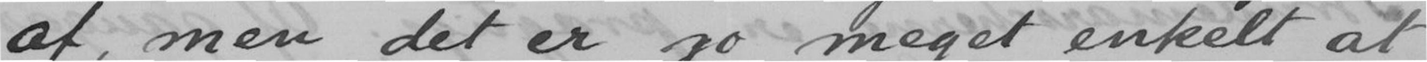af, men det er jo meget enkelt at
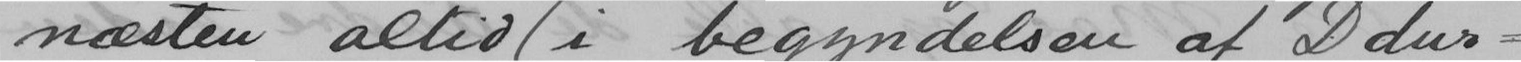næsten altid (i begyndelsen af Ddur-
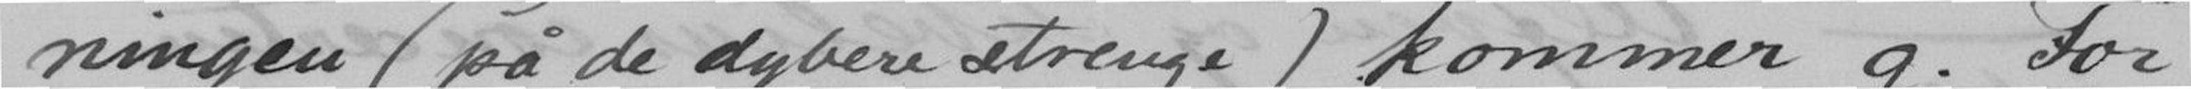ningen (på de dybere strenge) kommer g. For
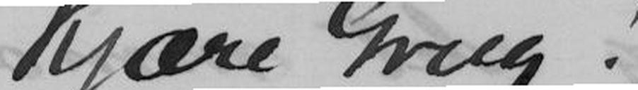Kjære Grieg!
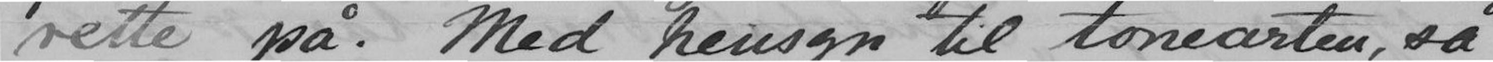rette på. Med hensyn til tonearten, så
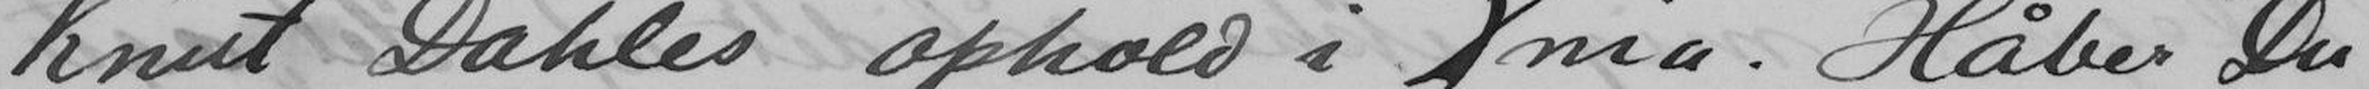Knut Dahles ophold i Xnia. Håber Du
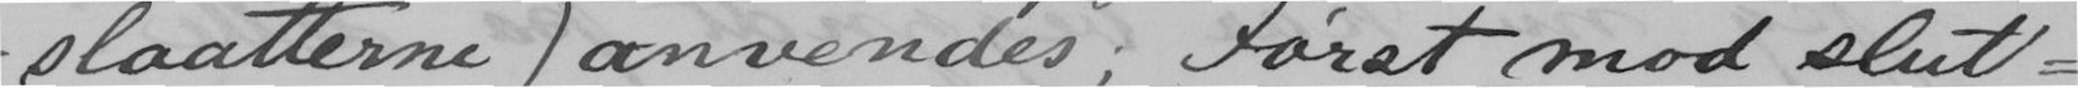slaatterne) anvendes; Først mod slut-
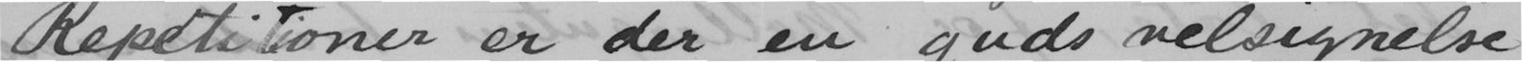Repetitioner er der en guds velsignelse
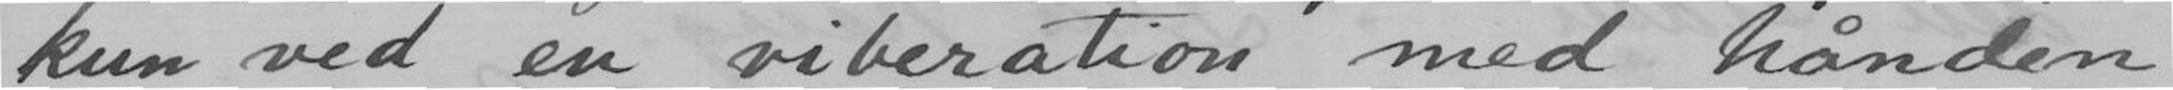kun ved en viberation med hånden
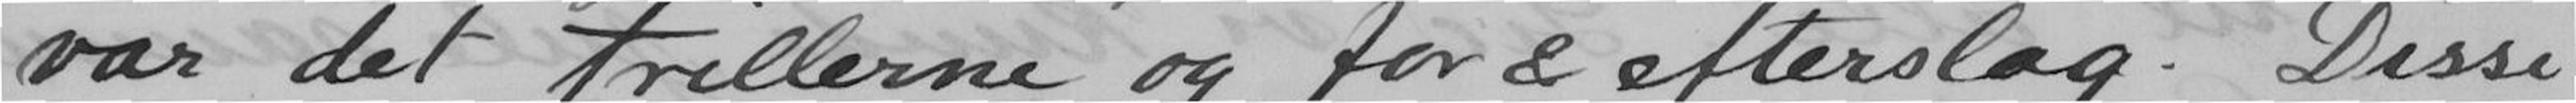var det trillerne og for & efterslag. Disse
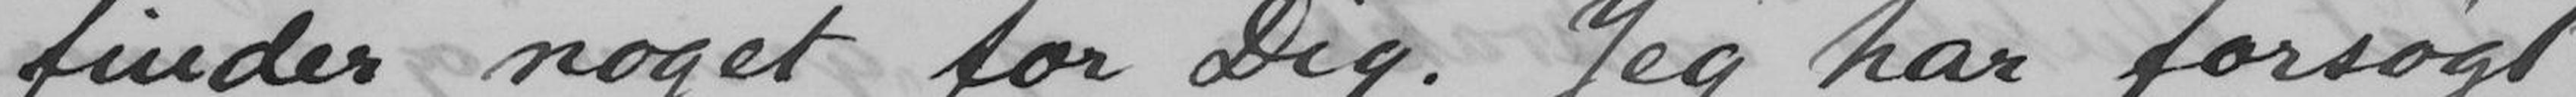finder noget for Dig. Jeg har forsøgt
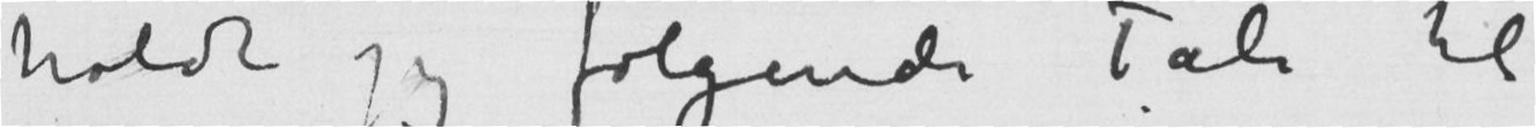holdt jeg følgende Tale til
